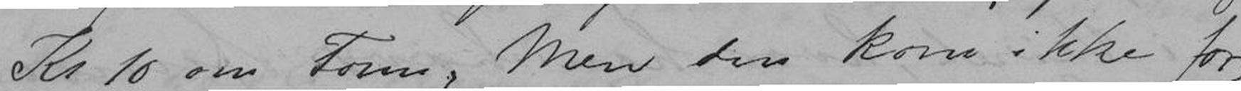Kl 10 om Form. Men den kom ikke for,
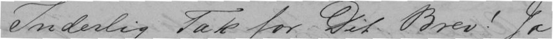Inderlig Tak for Dit Brev! Ja
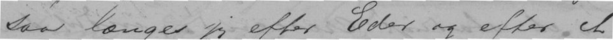saa længes jeg efter Eder og efter et
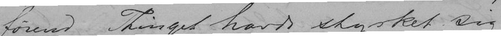førend Thinget havde styrket sig
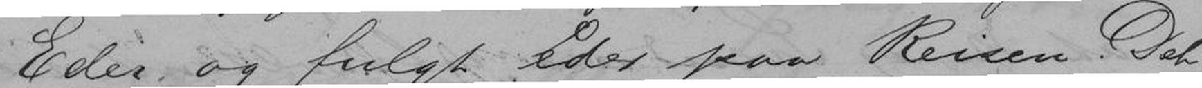Eder, og fulgt Eder paa Reisen. Det
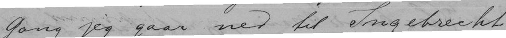Gang jeg gaar ned til Ingebrecht
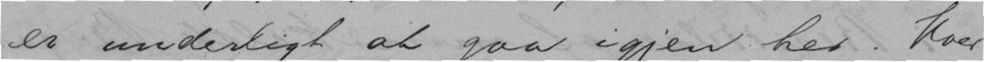er underligt at gaa igjen her. Hver
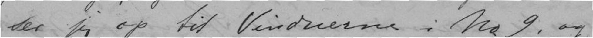ser jeg op til Vinduerne i N. 9, og
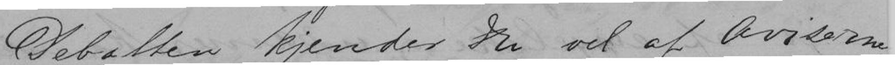Debatten kjender Du vel af Aviserne.
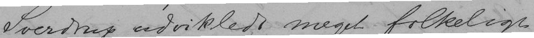Sverdrup udviklede meget folkeligt
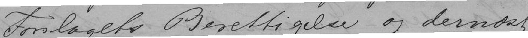Forslagets Berettigelse og dernæst
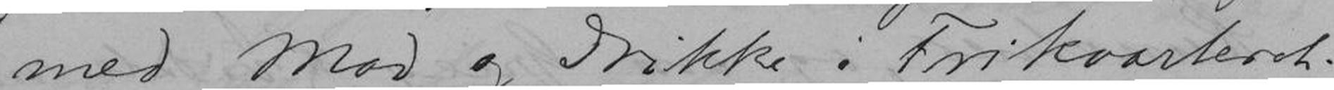med Mad og Drikke i Frikvarteret.
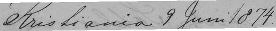Kristiania 9 Juni 1874.
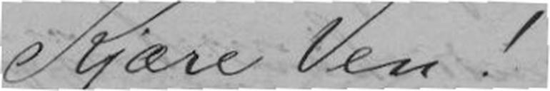Kjære Ven!
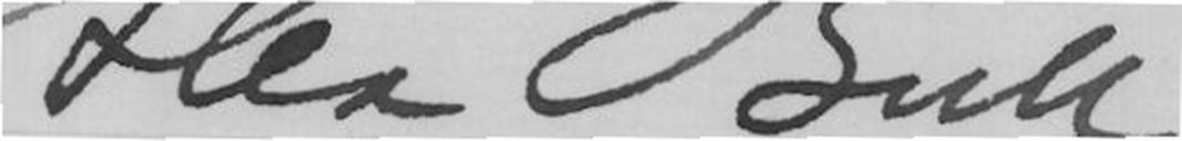Alex Bull
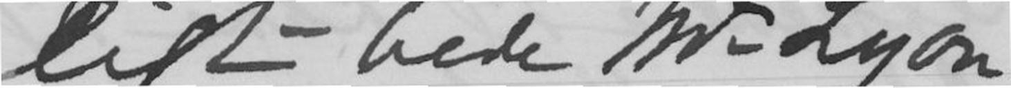ligt bede Mr Lyon
